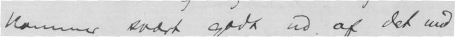kommer svært godt ud af det med
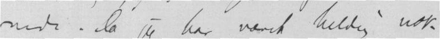nede. Da jeg har været heldig nok
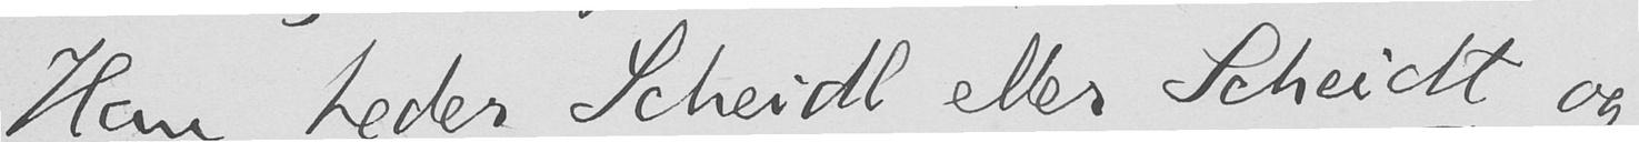Han heder Scheidl eller Scheidt og
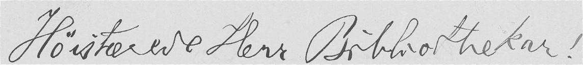Høistærede Herr Bibliothekar!
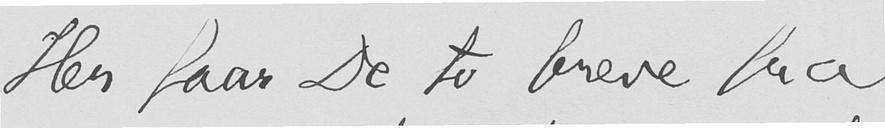Her faar De to breve fra
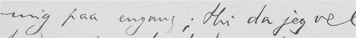mig paa engang; thi da jeg vel
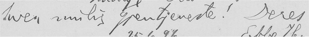hver mulig gjentjeneste! Deres
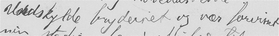Undskylde bryderiet og vær forvisset
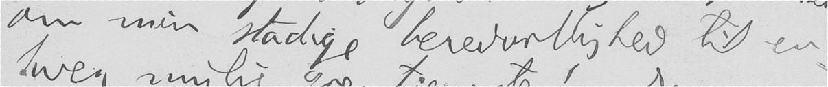om min stadige beredvillighed til en
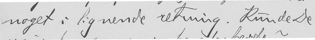noget i lignende retning. Kunde De
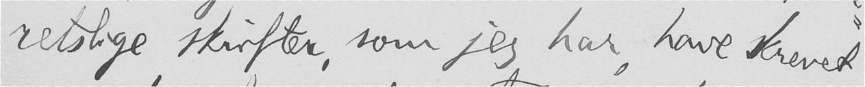retslige skrifter, som jeg har, have skrevet
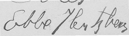Ebbe Hertzberg.
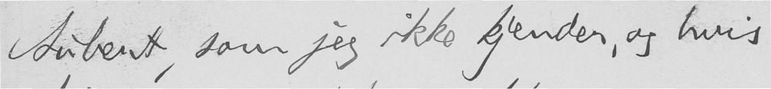Aubert, som jeg ikke kjender, og hvis
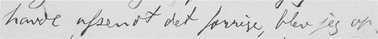havde afsendt det forrige, blev jeg op-
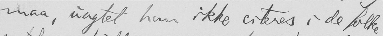maa, uagtet han ikke citeres i de folke-
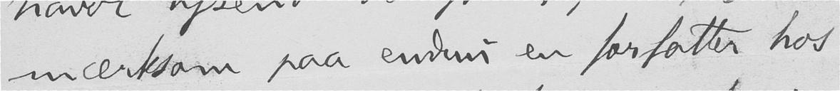mærksom paa endnu en forfatter hos
